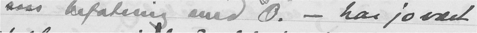sin befatning med O. - har jo vært
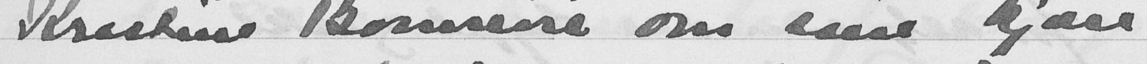Kristine Bonnevie om sine kjære
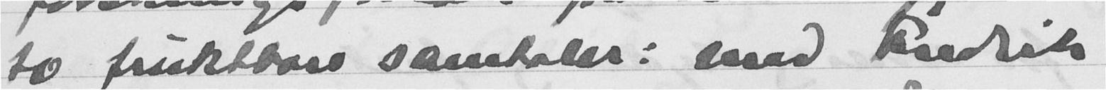to fruktbare samtaler; med Fredrik
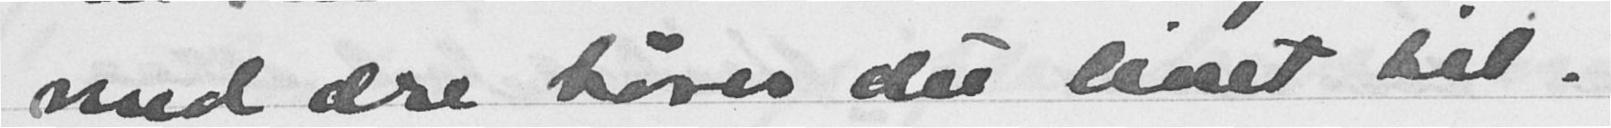med ære hører du livet til.
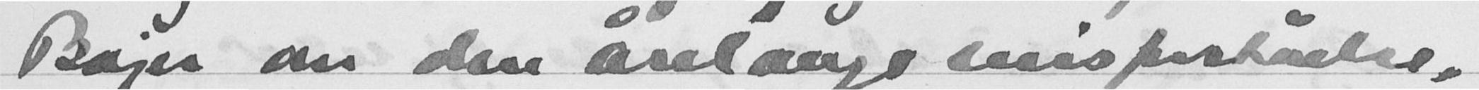Bojer om den årelange misforståelse,
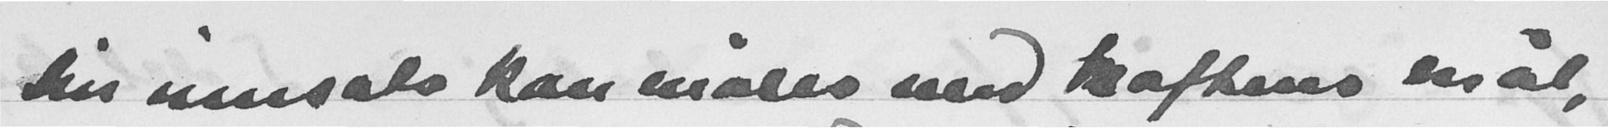Din innsats kan måles med kaftens mål,
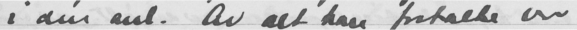i den anl. Av alt han fortalte var
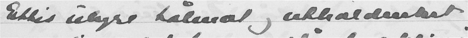Ettis uhyre tålmot og utholdenhet
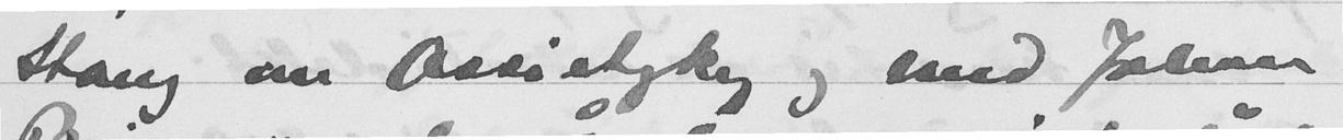Stang om Ossietzky og med Johan
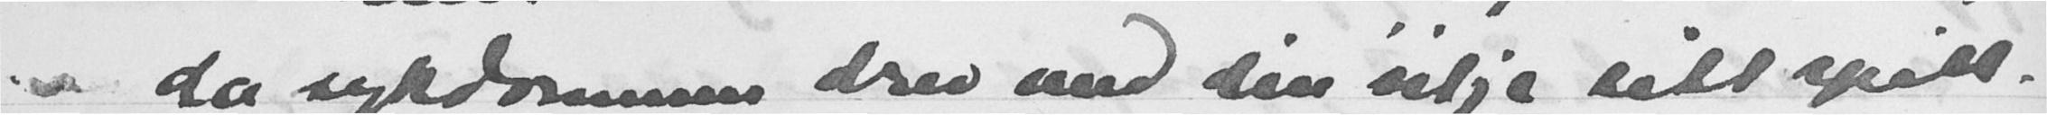da sykdommen drev med din vilje sitt spill.
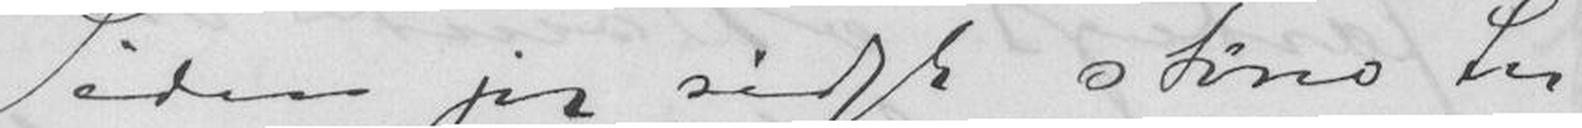Siden jeg sidst skrev til
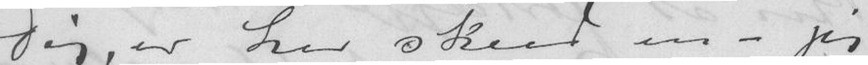Dig, er her skeed en - jeg
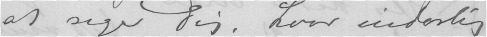at sige Dig, hvor inderlig
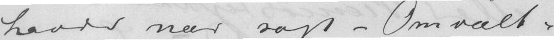havde nær sagt - Omvælt-
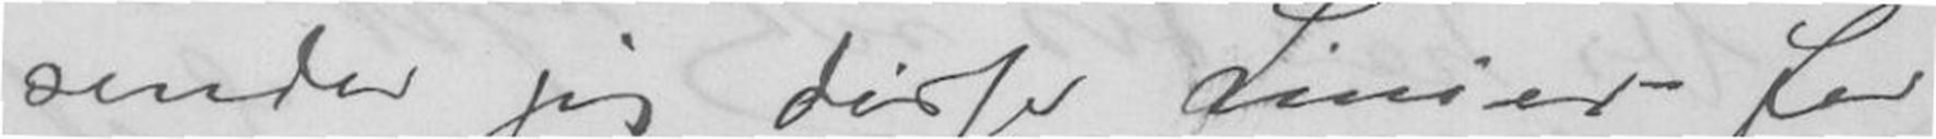sender jeg disse Linier for
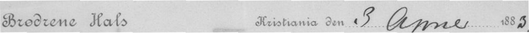Brødrene Hals Kristiania den 3 April 1883.
